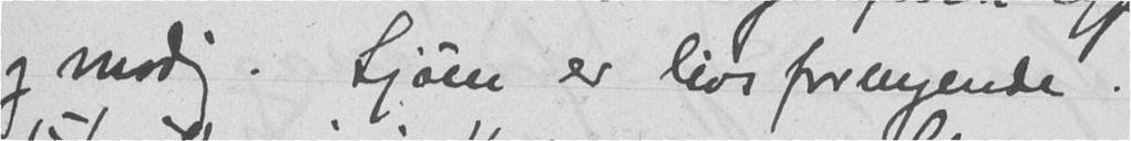og modig. Sjøen er livsfornyende.
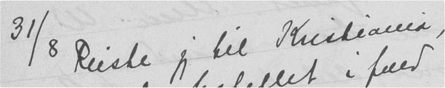31/8 Reiste jeg til Kristiania,
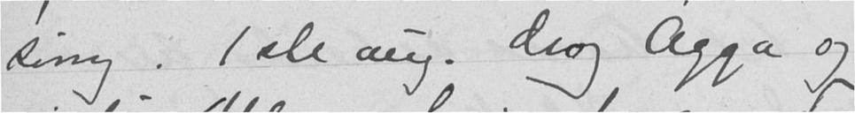sving. 1ste aug. drog Agga og
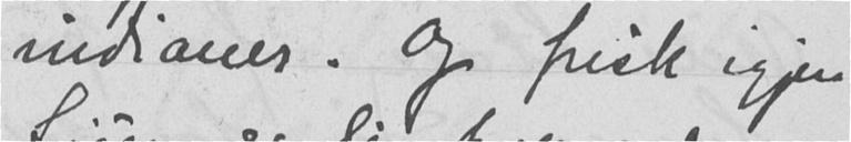indianer. Og frisk igjen
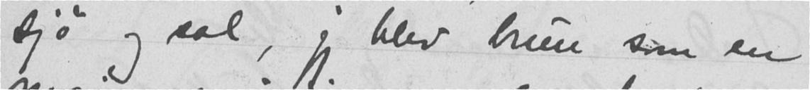sjø og sol, jeg blev brun som en
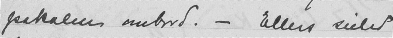pokalen ombord. - Ellers seiled
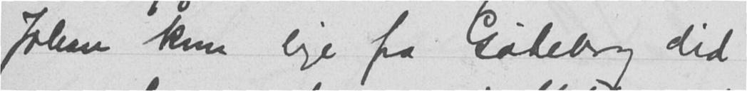Johan kom lige fra Gøteborg did
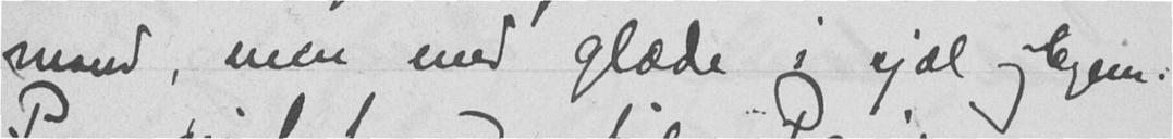mand, men med glæde i sjæl og hjem.
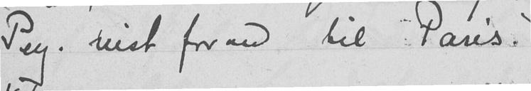Pey. reist for ned til Paris.
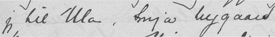jeg til Ula, Sonja Nygaard
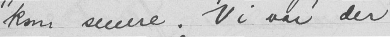kom senere. Vi var der
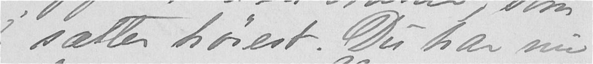jeg sætter høiest. Du har nu
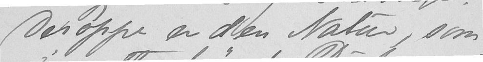Deroppe er den Natur, som
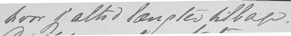hvor jeg altid længter tilbage.
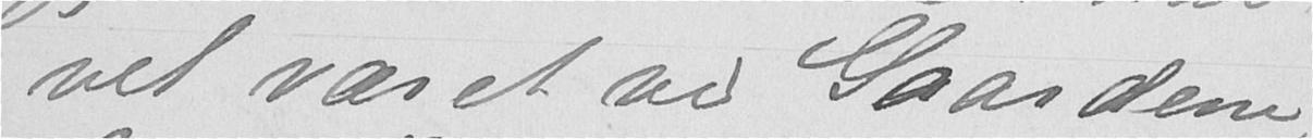vel været ved Gaardene
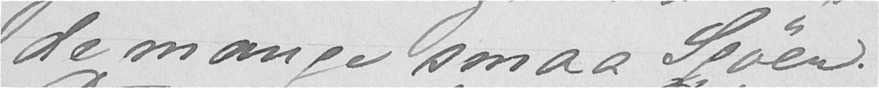de mange smaa Sjøene.
